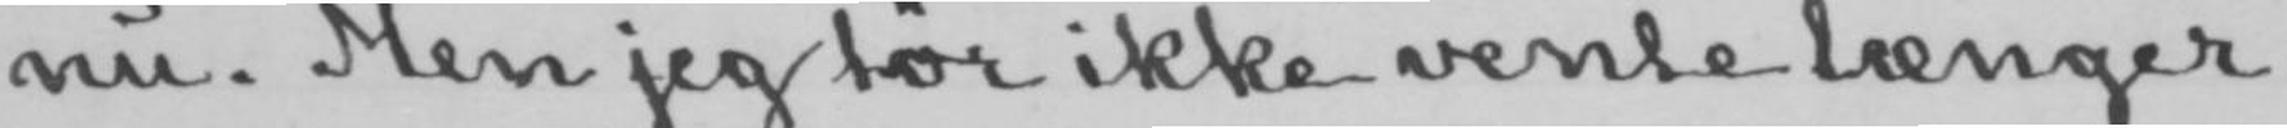nu. Men jeg tør ikke vente længer
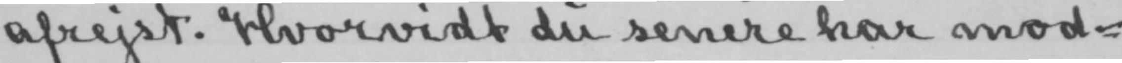afrejst. Hvorvidt du senere har mod-
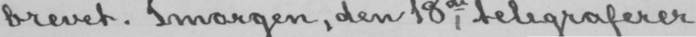brevet. Imorgen, den 18de, telegraferer
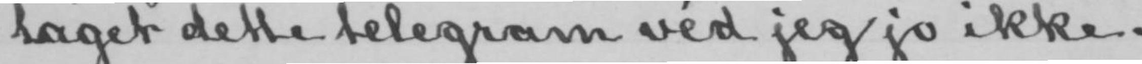taget dette telegram véd jeg jo ikke.
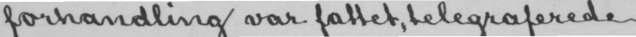forhandling var fattet, telegraferede
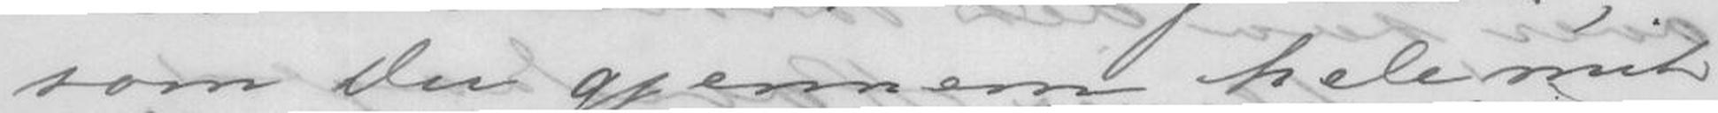som Du gjennem hele mit
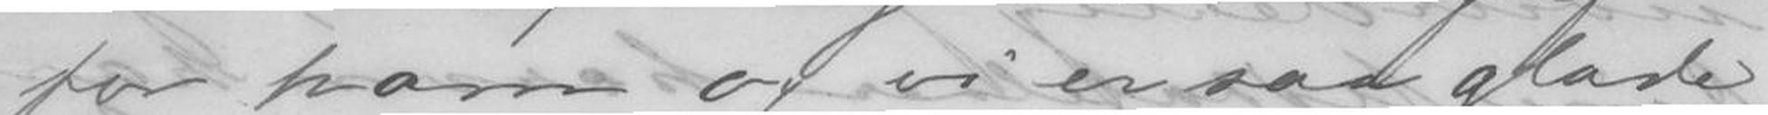for ham og vi er saa glade
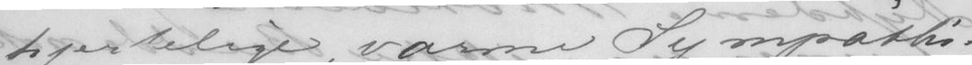hjertelige, varme Sympathi.
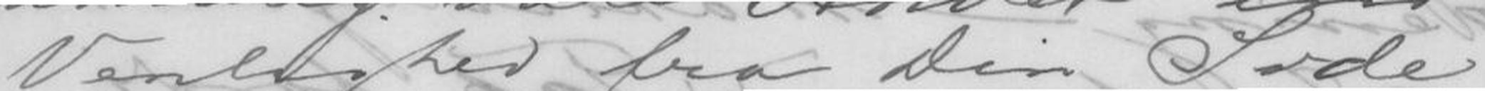Venlighed fra Din Side
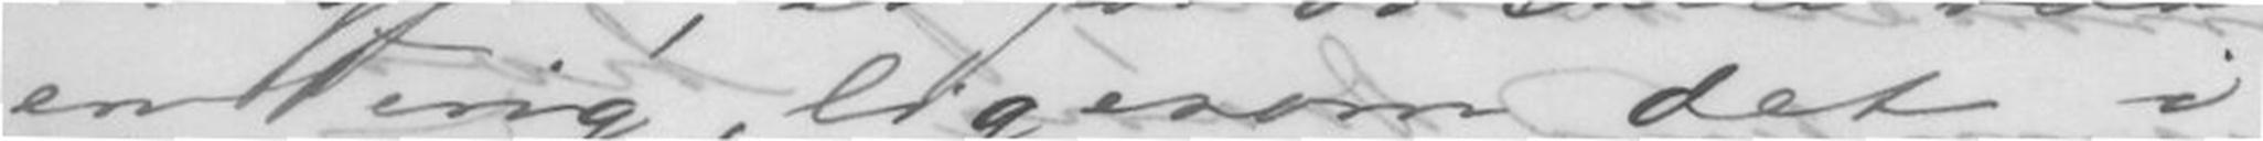en Ting, ligesom det i
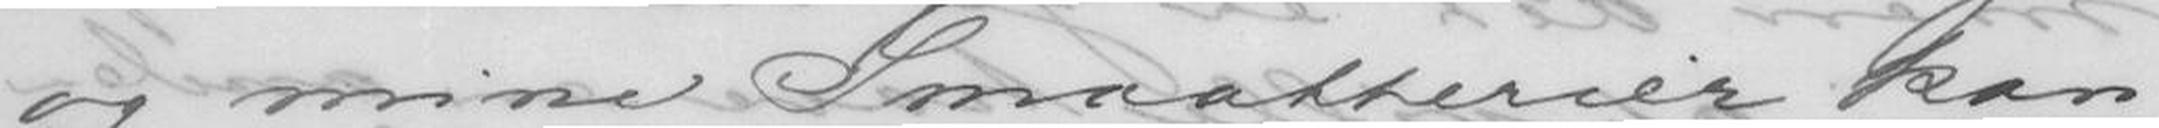og mine Smaatterier kan
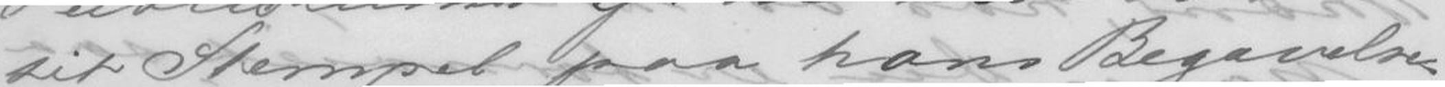sit Stempel paa hans Begavelse.
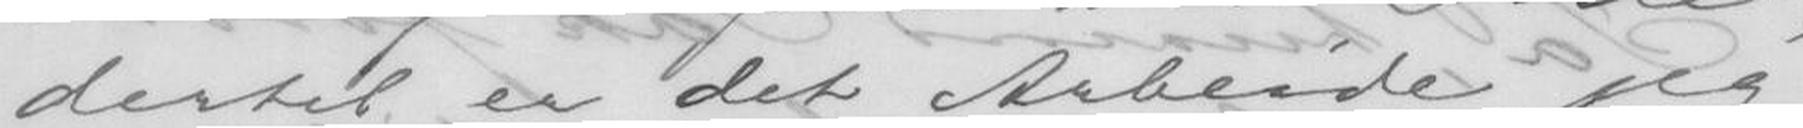dertil er det Arbeide jeg
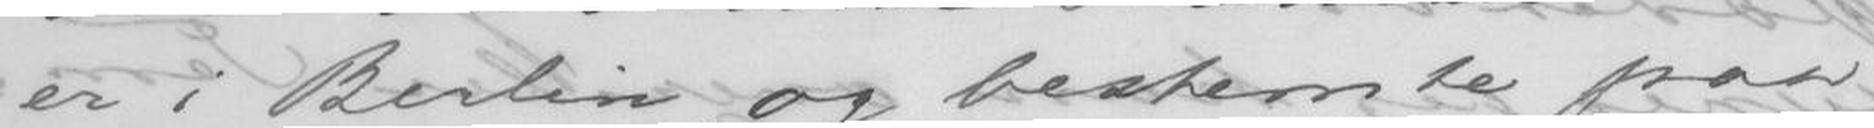er i Berlin, og bestemte paa
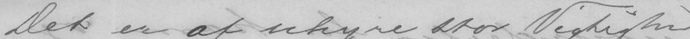Det er af uhyre stor Vigtighed
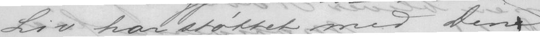Liv har støttet med Din
